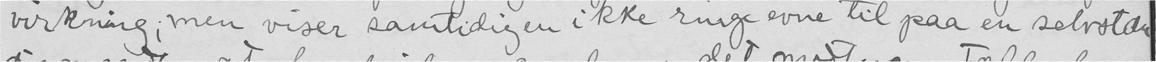virkning, men viser samtidigen ikke ringe evne til paa en selvstæn-
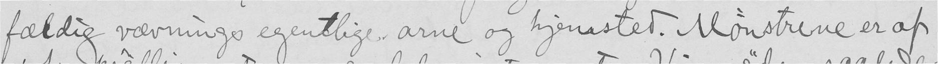fældig vævnings egentlige arne og hjemsted. Mønstrene er af
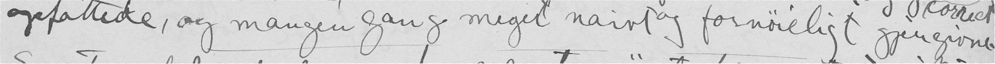opfattede, og mangen gang meget naivt og fornøieligt gjengivne.
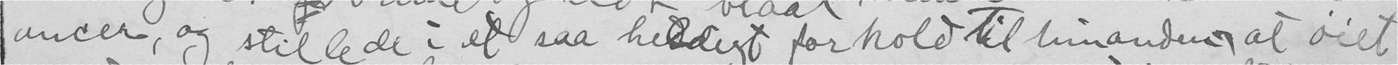ancer, og stillede i et saa heddigt forhold til hinanden at øiet
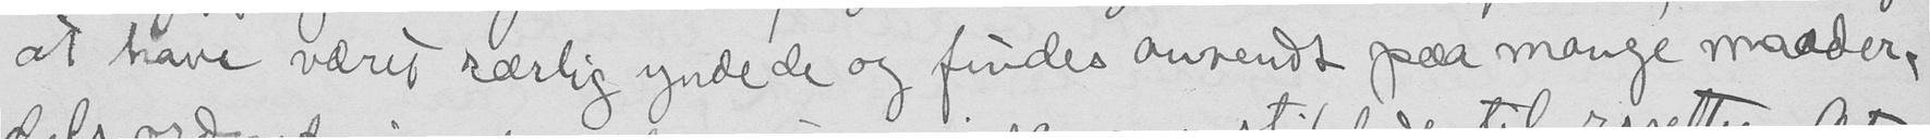at have været særlig yndede og findes anvendt paa mange maader,
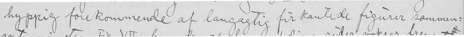hyppig forekommende af langagtig firkantede figurer sammen-
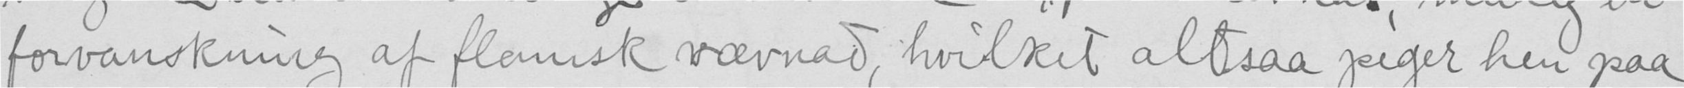forvanskning af flemsk vævnad, hvilket altsaa peger hen paa
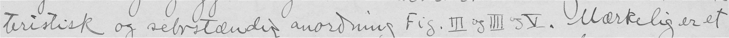teristisk og selvstændet anordning Fig. III og IIII og V. Mærkelig er et
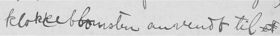klokkeblomsten omvendt til
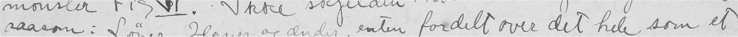saasom: Løven, Haren og anden, enten fordelt over det hele som et
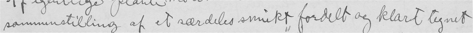sammenstilling af et særdeles smukt fordelt og klart tegnet
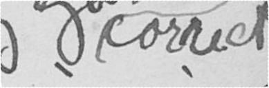correct
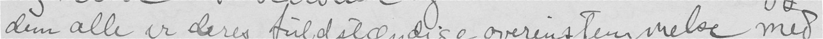dem alle er deres fuldstændige overeinstemmelse med
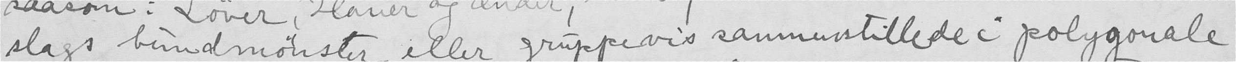slags bundmøtster eller grunpevis sammenstillede i polygmale
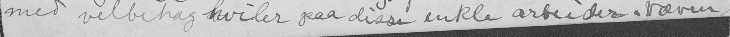med velbehag hviler paa disse enkle arbeider-væven
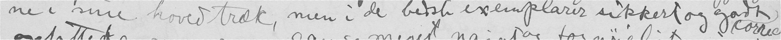ne i sine hovedtræk, men i de bedste exemplarer sikkert og godt
